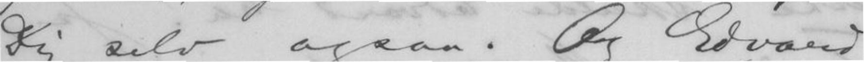Dig selv ogsaa. Og Edvard,
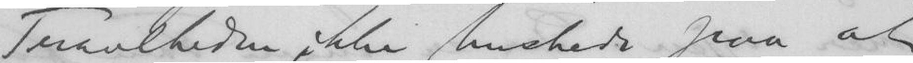Travlheden ikke huskede paa at
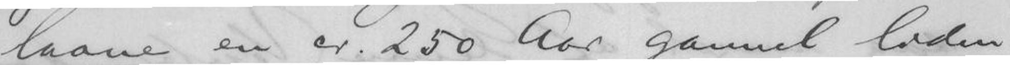laane en ca. 250 Aar gammel liden
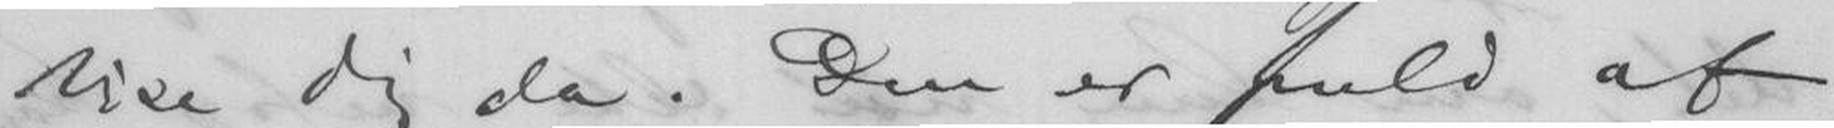vise dig da. Den er fuld af
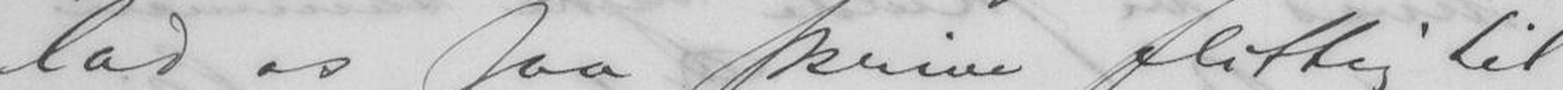lad os saa skrive flittig til
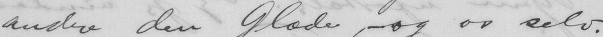andre den Glæde, - og os selv.
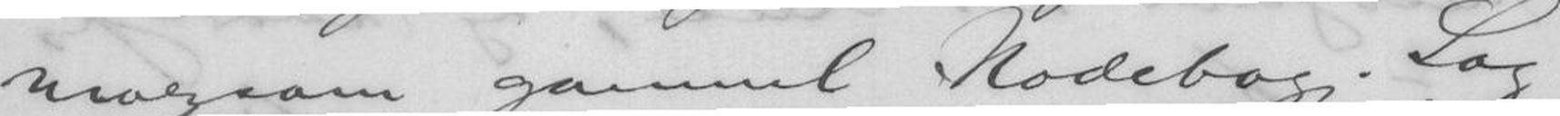morsom gammel Nodebog. Log-
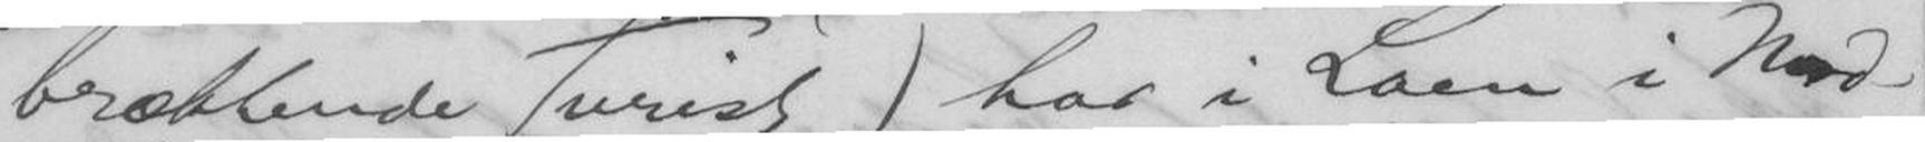brækkende Turist) har i Loen i Nord-
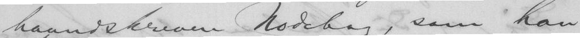haandskreven Nodebog, som han
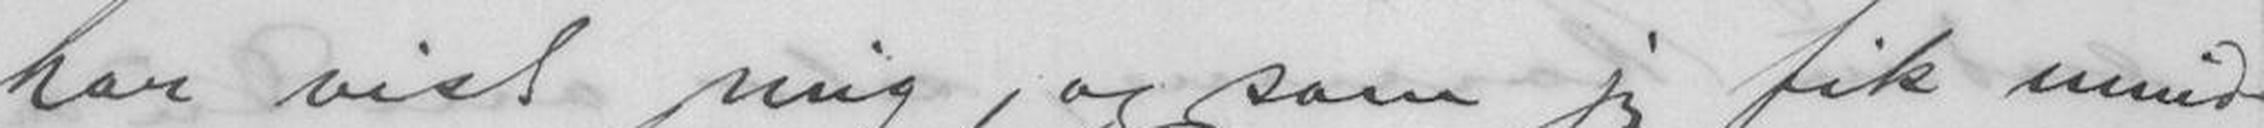har vist mig, og som jeg fik umid-
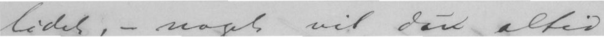lidet, - noget vil du altid
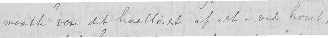maatte være det haabløseste af alt - ved havet,
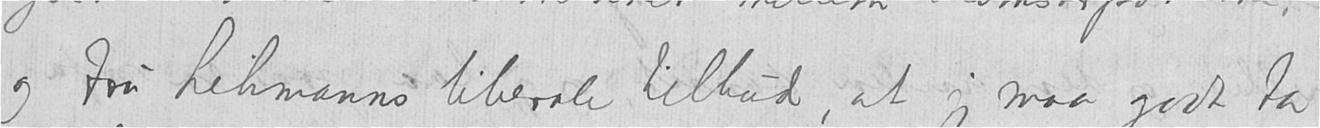og Fru Lehmanns liberale tilbud, at jeg maa godt ta
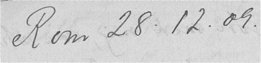Rom 28.12.09.
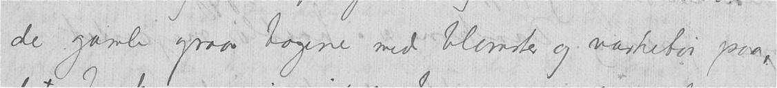de gamle graa tagene med blomster og vasketøi paa,
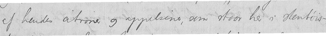af hendes citroner og appelsiner, som staar her i stentøis-
